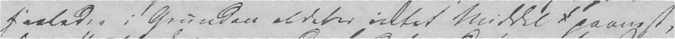saaledes i Grunden aldeles intet Middel paavist,
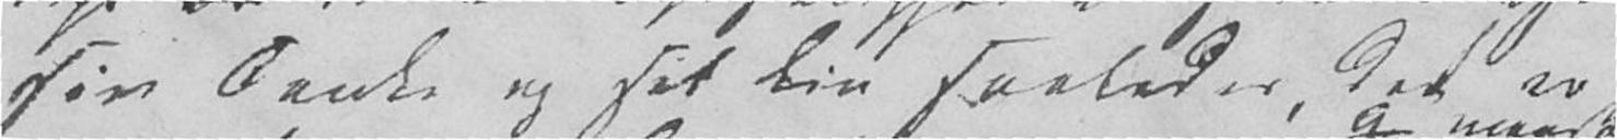sin Tanke og sit Liv saaledes, det er
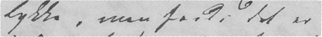Lykke, men fordi det er
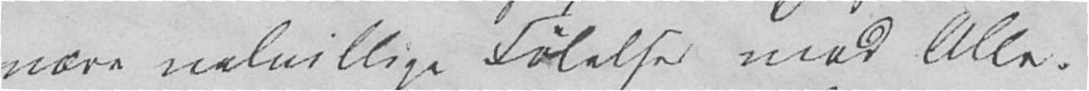nære velvillige Følelser mod Alle.
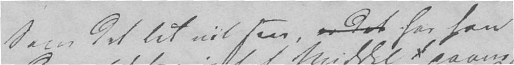Som det let vil sees, er det har han
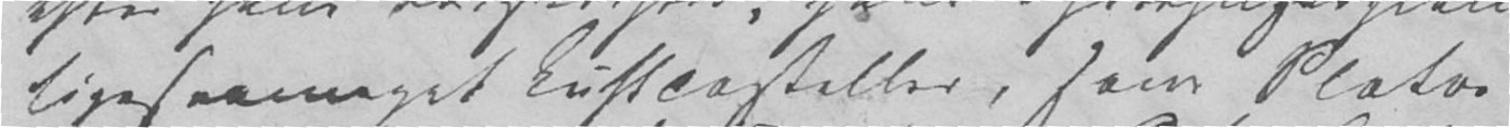ligesaameget Luftcasteller, som Platos
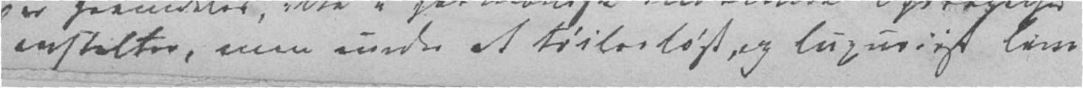anstalter, men under et tøilesløst og luxuriøst Livs
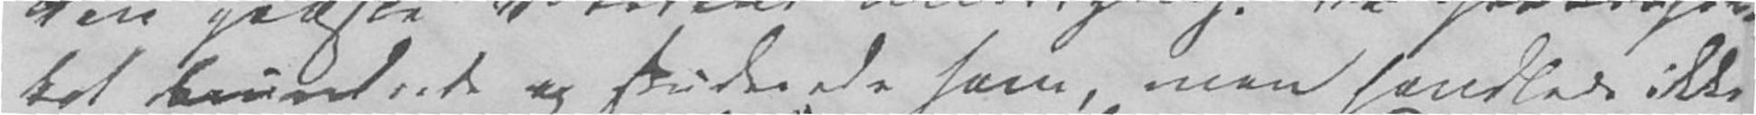ket beundrede og studerede ham, men handlede ikke
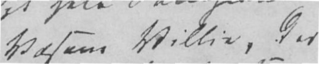Væsens Villie, der
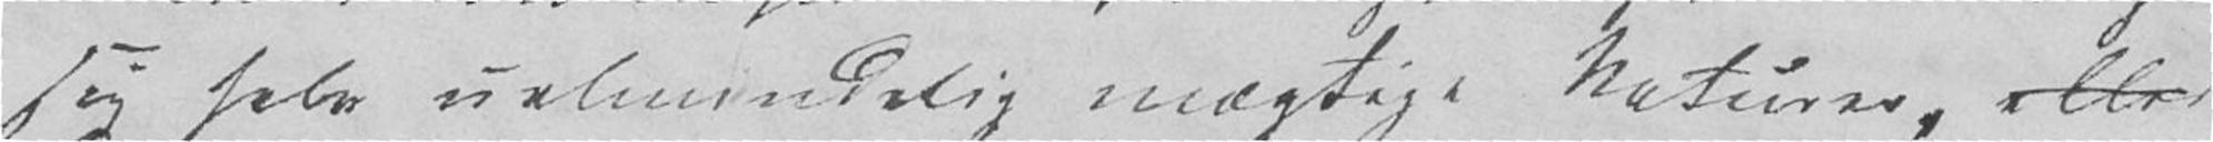sig selv ualmindelig mægtige Naturer, eller
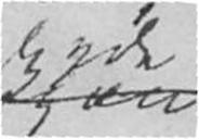byde
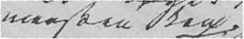maaske kan
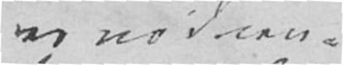er nødven-
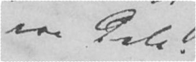er Dele.
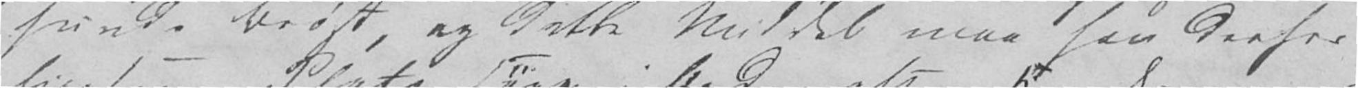funds Brøst, og dette Middel, maa han derfor
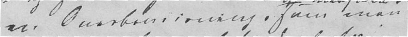en Overbeviisning, som man
